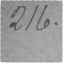216.
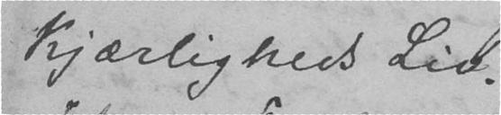Kjærligheds Liv.
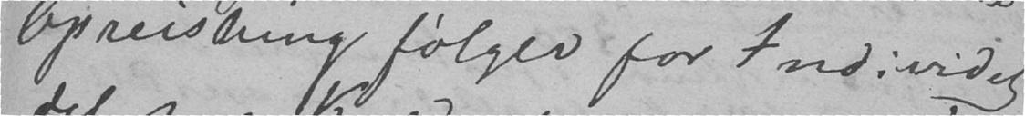Opreisning følger for Individet
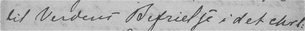til Verdens Befrielse i det chrl:
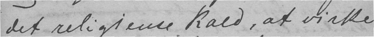det religieuse Kald, at virke
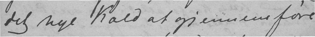det nye Kald at gjennemføre
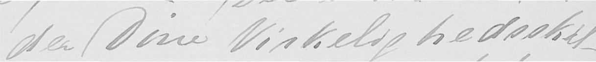der Dine Virkelighedsskil-
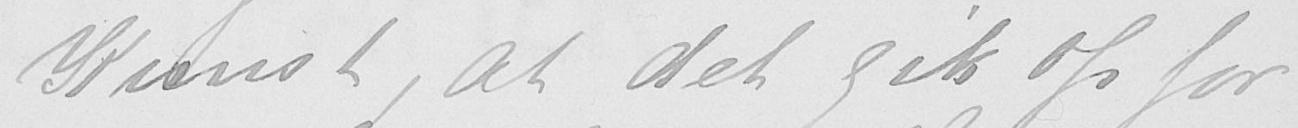Kunst, at det gik op for
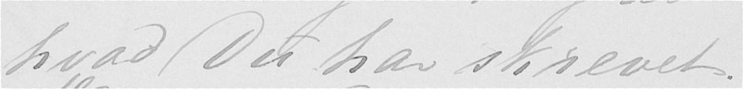hvad Du har skrevet.
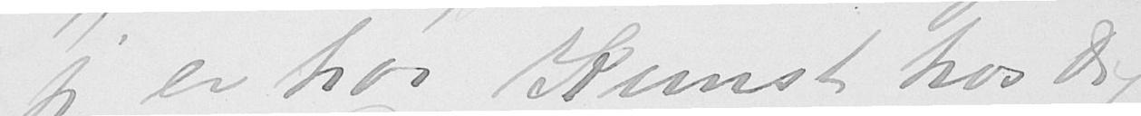jeg er høi Kunst hos Dig,
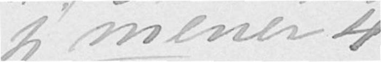jeg mener 4
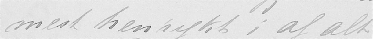mest henrykt i af alt
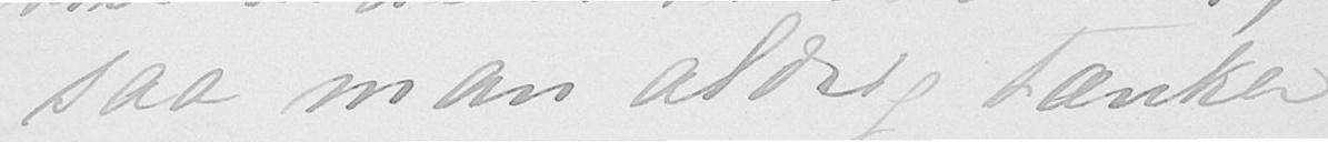saa man aldrig tænker
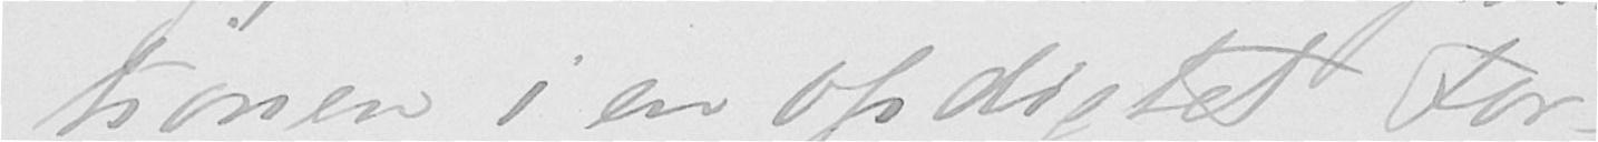tionen i en opdigtet For-
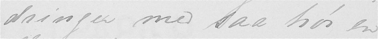dringer med saa høi en
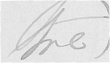tre
